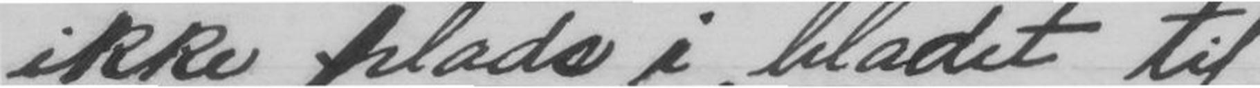ikke plads i bladet til
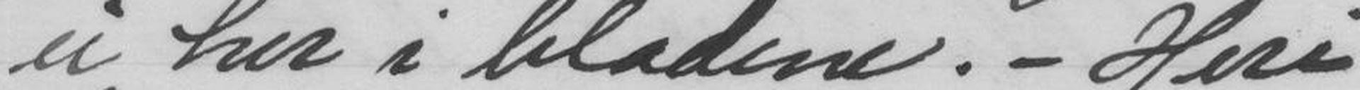ei her i bladene. - Heri
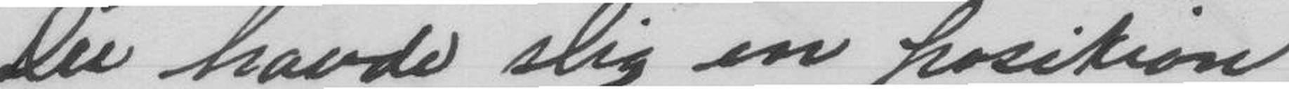Du havde slig en position
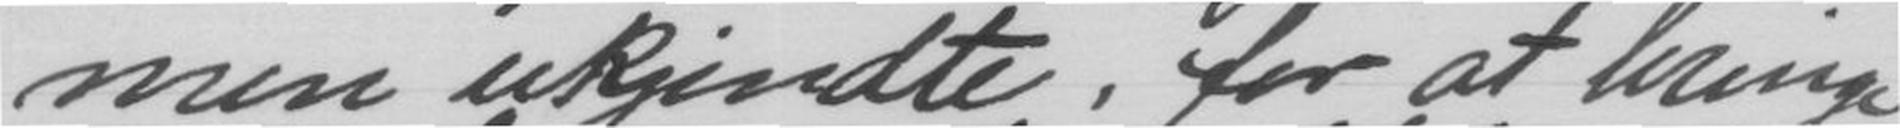men ukjendte, for at bringe
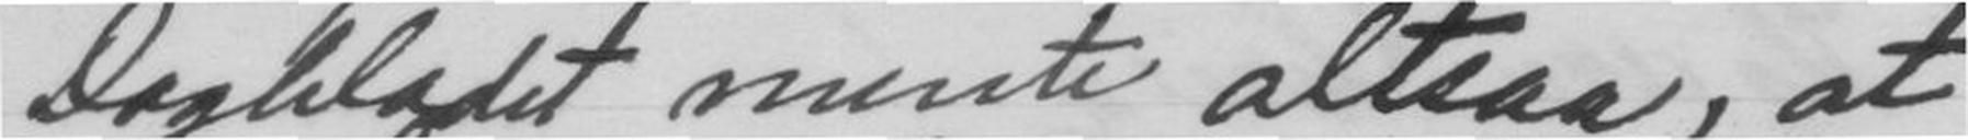Dagbladet mente altsaa, at
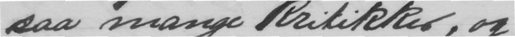saa mange kritiker, og
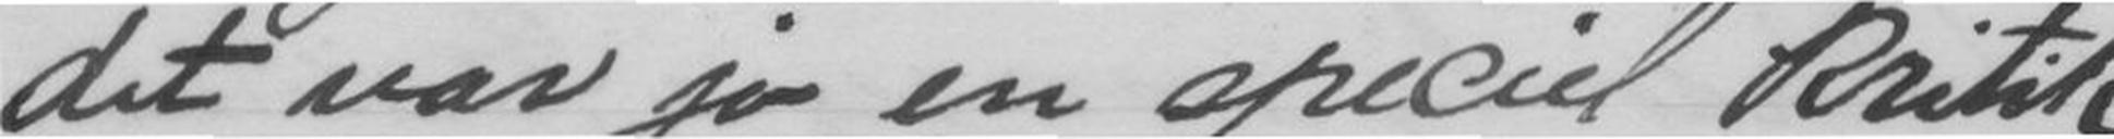det var jo en spiciel kritik
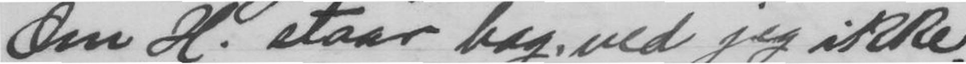Om H. staar bag, ved jeg ikke
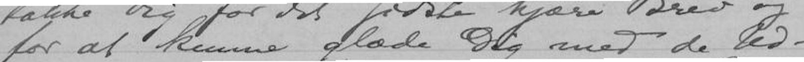for at kunne glæde Dig med de Ud-
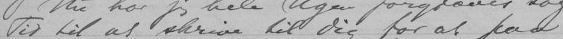Tid til at skrive til Dig for at faa
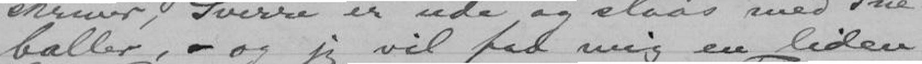baller, - og jeg vil faa mig en liden
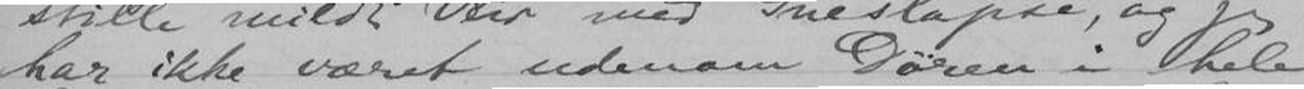har ikke været udenom Døren i hele
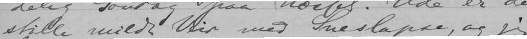stille mildt Veir med Sneslapse, og jeg
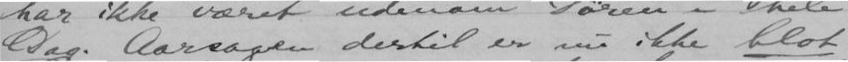Dag. Aarsagen dertil er nu ikke blot
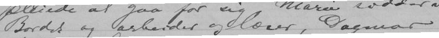Bordet og arbeider og læser, Dagmar
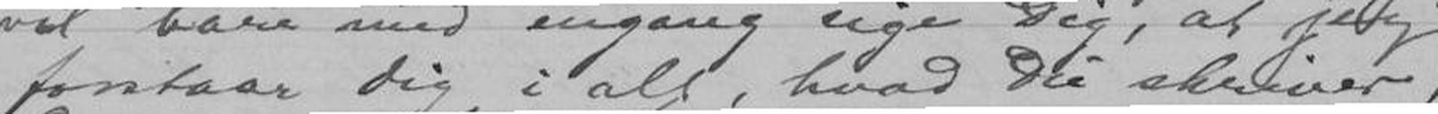forstaar Dig i alt, hvad Du skriver,
